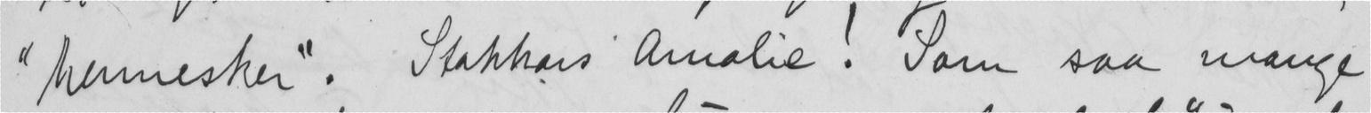"Mennesker". Stakkars Amalie! Som saa mange
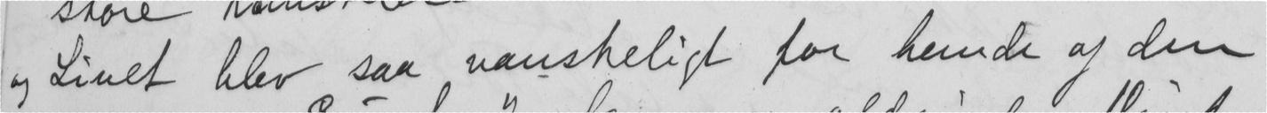og Livet blev saa vanskeligt for hende og den
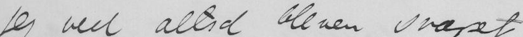Jeg ved altid bleven svaret
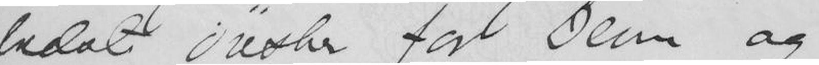bedste ønsker for Dem og
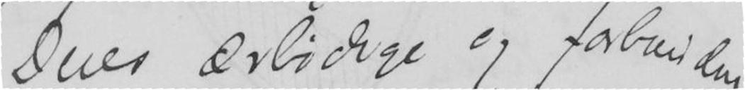Deres ærbødige og forbundne
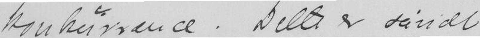konkurrance. Dette er såndt
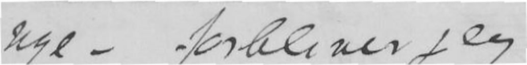nye - forbliver jeg
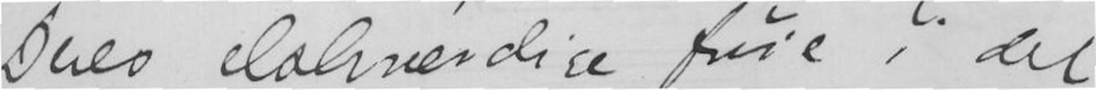Deres elskværdige frue i det
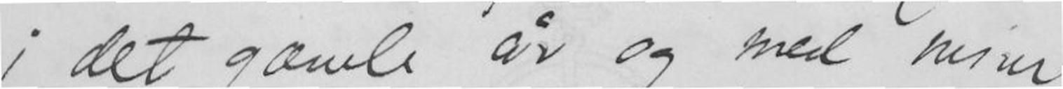i det gamle år og med mine
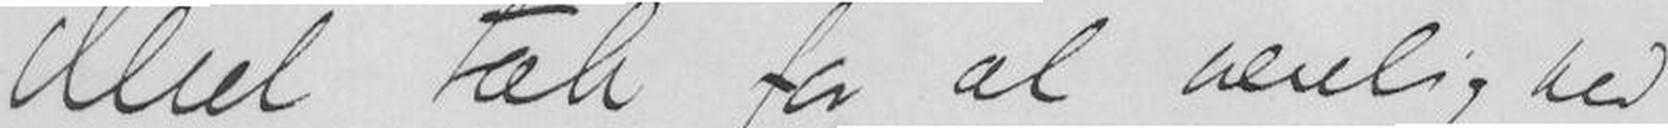Med Tak for al venlighed
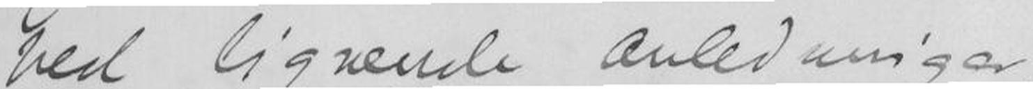ved lignende Anledningar.
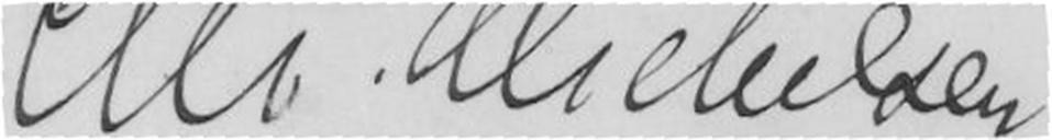Chr. Michelsen
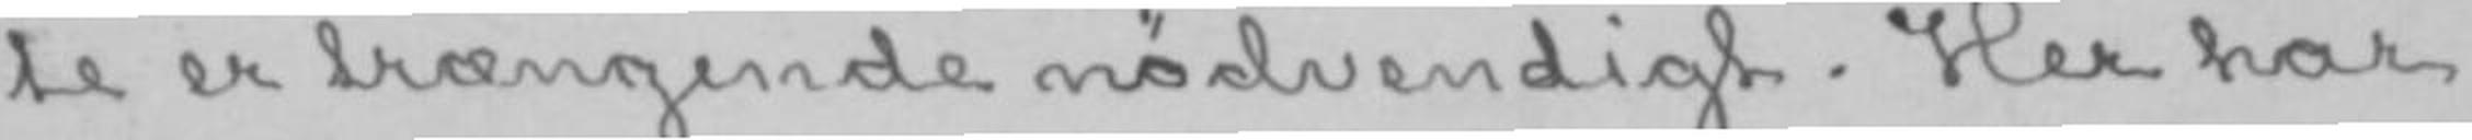te er trængende nødvendigt. Her har
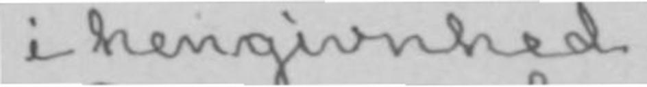i hengivenhed
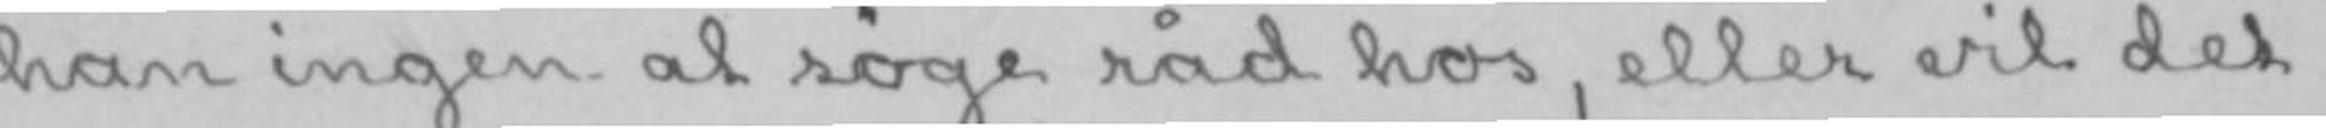han ingen at søge råd hos, eller vil det
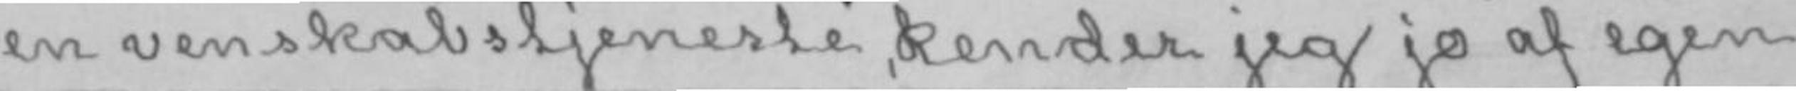en venskabstjeneste, kender jeg jo af egen
